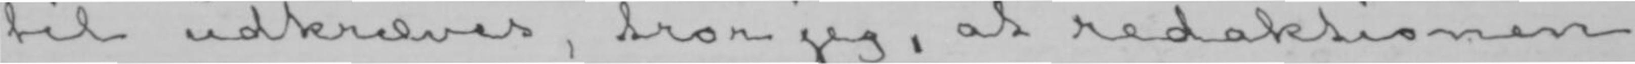til udkræves, tror jeg, at redaktionen
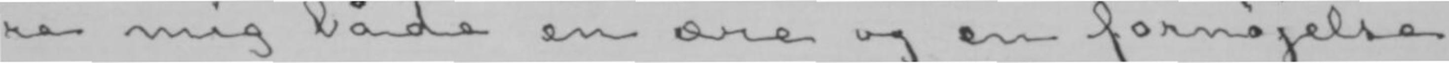re mig både en ære og en fornøjelse
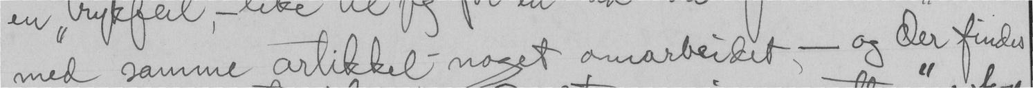med samme artikkel noget omarbeidet, - og der findes
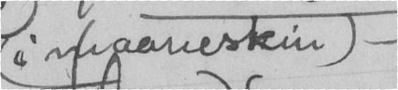(i maaneskin)
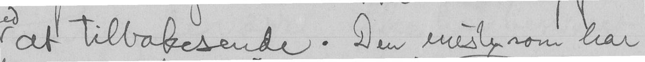at tilbakesende. Den eneste som har
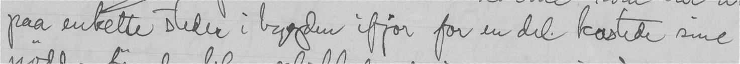paa enkette steder i bygden ifjor for en del kostete sine
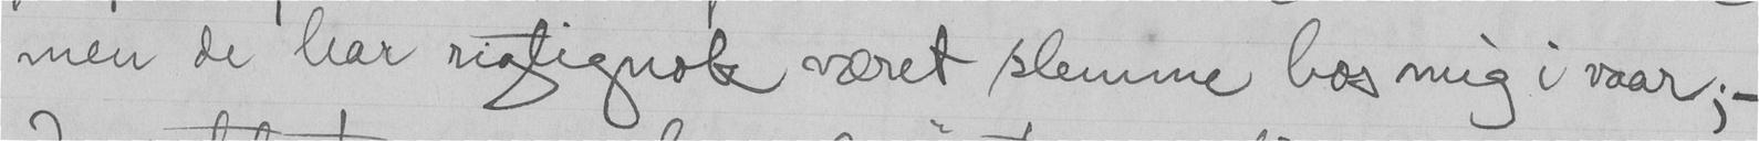men de har rigtignok været slemme hog mig i vaar; -
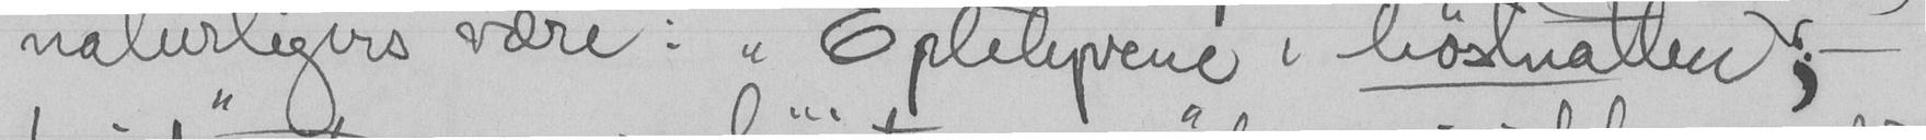naturligvis være : "Epletyvene i høstnatten); -
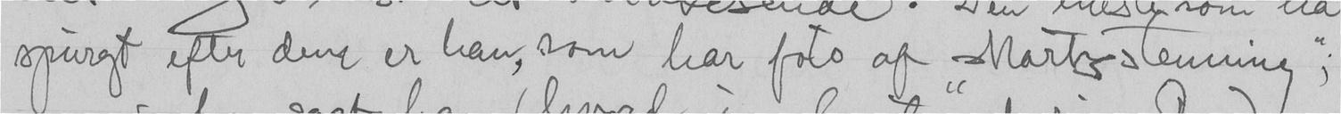spurgt efter den er han, som har foto at "Martzstemning";
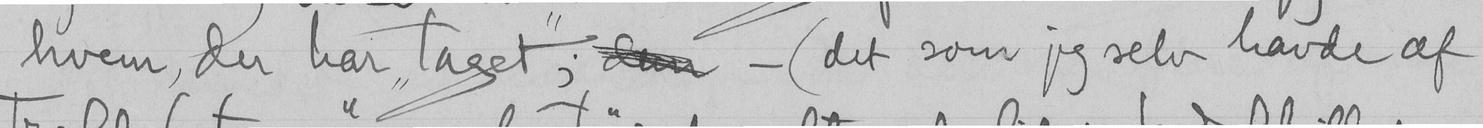hvem, der har "taget";  - (det som jeg selv havde af
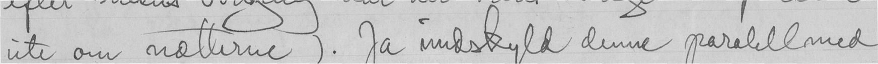ute om nætterne). Ja undskyld denne paralell med
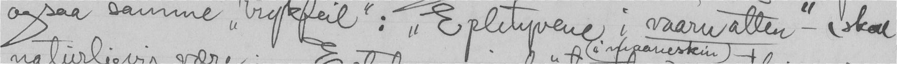ogsaa samme "trykfeil": "Epletyvene i vaarnatten" - (skal
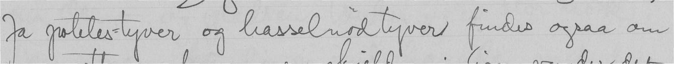Ja potetes-tyver og hasselnødtyver findes ogsaa om
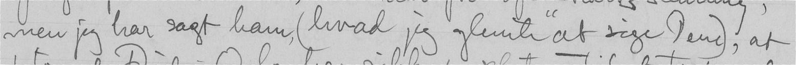men jeg har sagt ham (hvad, og glemte at sige Dem); at
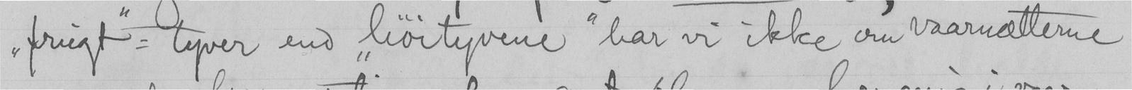"frugt" - tyver end "høityvene" har vi ikke om vaarnætterne,
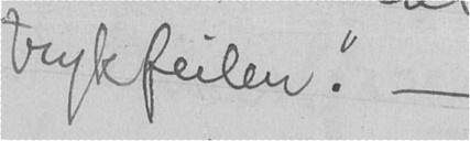"trykkfeilen". -
